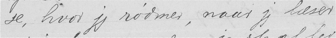se, hvor jeg rødmer, naar jeg læser
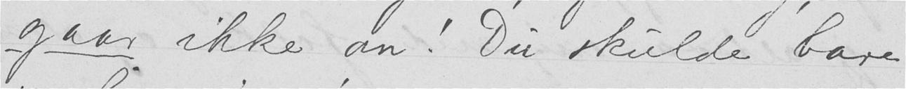gaar ikke an! Du skulde bare
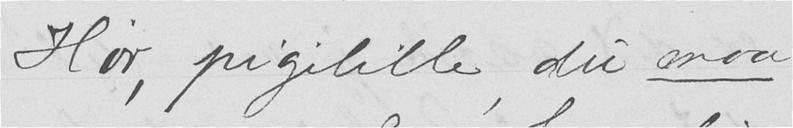Hør, pigelille du maa
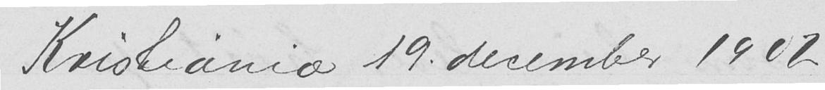Kristiania 19. december 1902
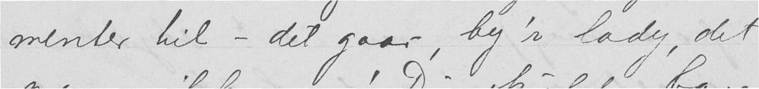menter til - det gaar, by'r lady, det
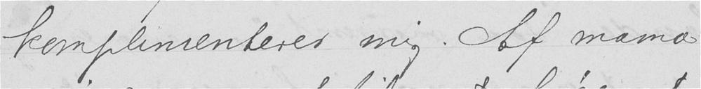komplimenterer mig. Af mama
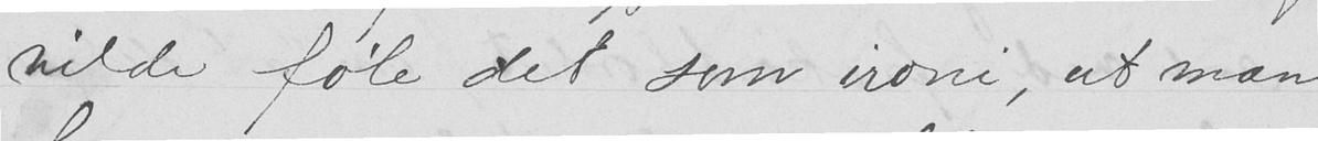vilde føle det som ironi, at man
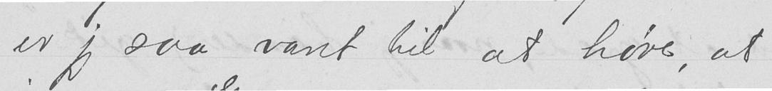er jeg saa vant til at høre, at
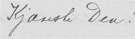Kjæreste Dea!
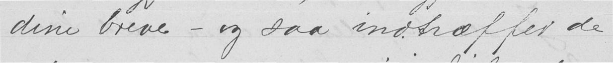dine breve - og saa indtræffer de
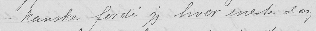- kanske fordi jeg hver eneste dag
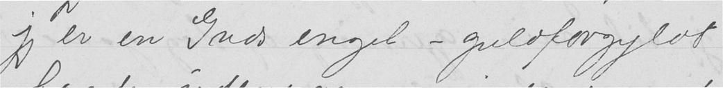jeg er en Guds engel - guldforgyldt
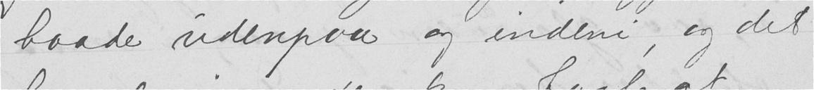baade udenpaa og indeni, og det
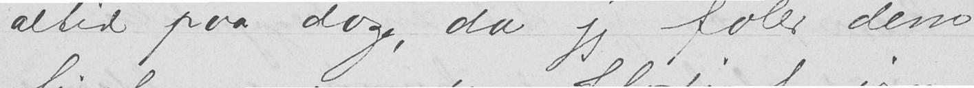altid paa dage, da jeg føler dem
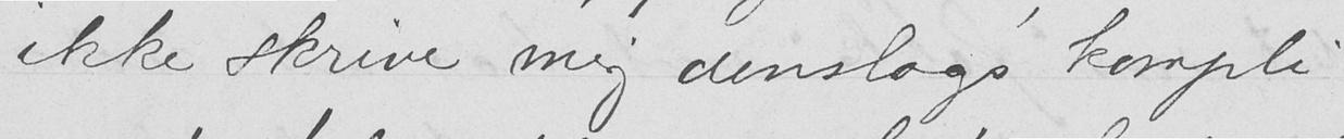ikke skrive mig denslags kompli-
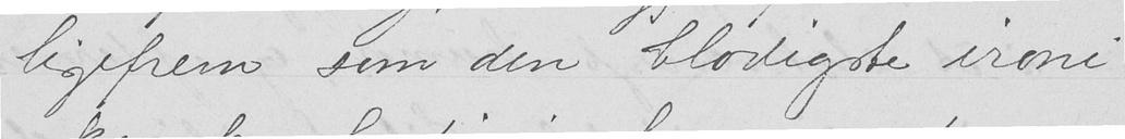ligefrem som den blodigste ironi
